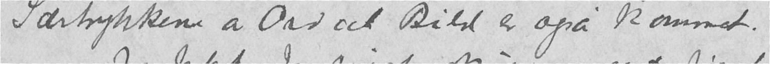Særtrykkene av Ord och Bild er også kommet.
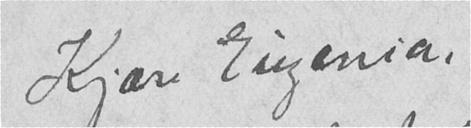Kjære Eugenia,
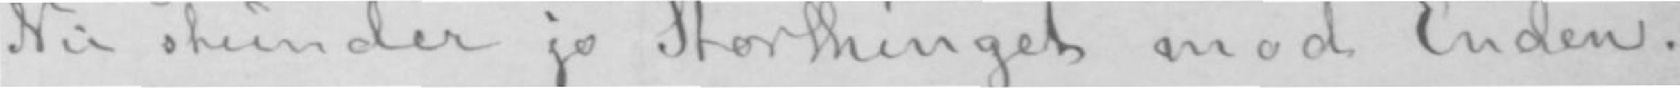Nu stunder jo Storthinget mod Enden.
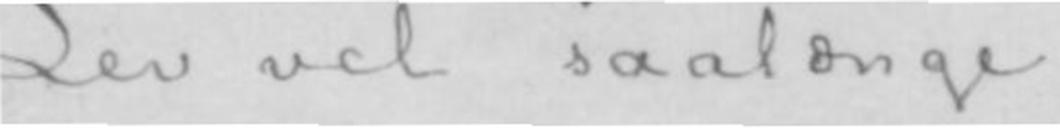Lev vel saalænge!
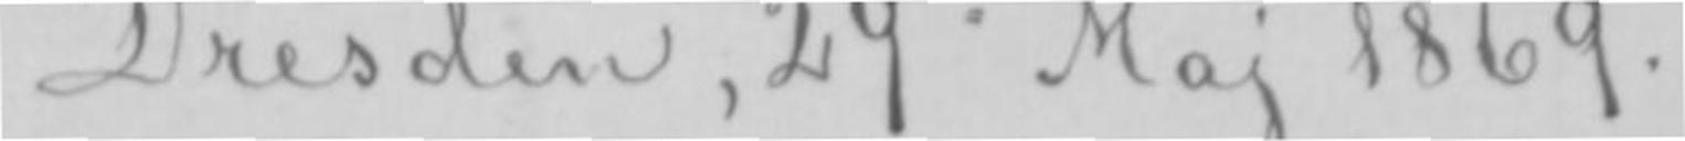Dresden, 29de Maj 1869.-
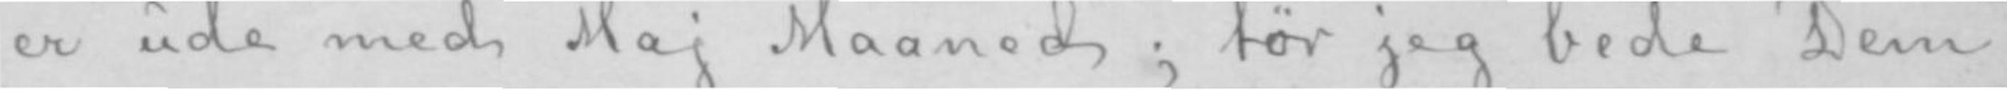er ude med Maj Maaned; tør jeg bede Dem
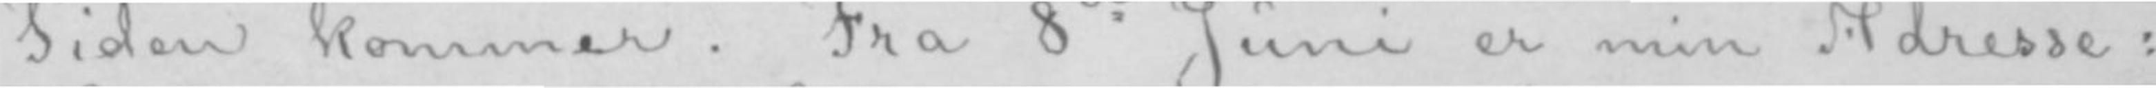Tiden kommer. Fra 8de Juni er min Adresse:
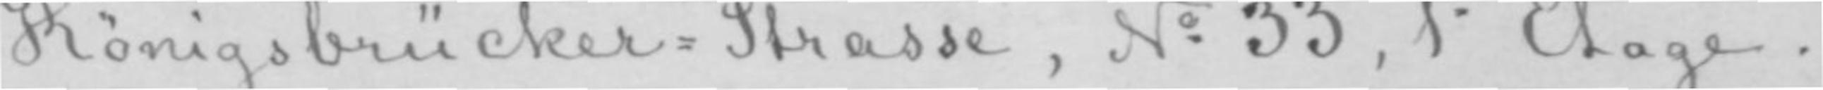«Königsbrücker-Strasse, No 33, 1te Etage.»
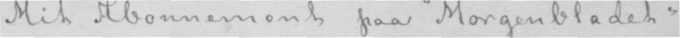Mit Abonnement paa «Morgenbladet»
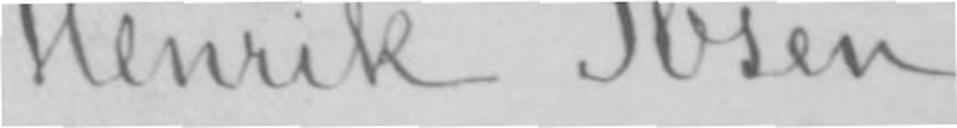Henrik Ibsen.
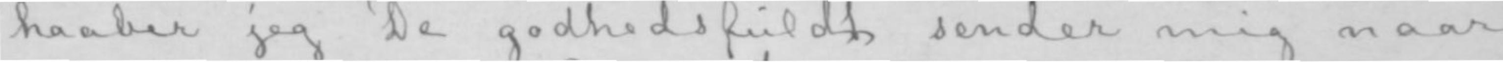haaber jeg De godhedsfuldt sender mig naar
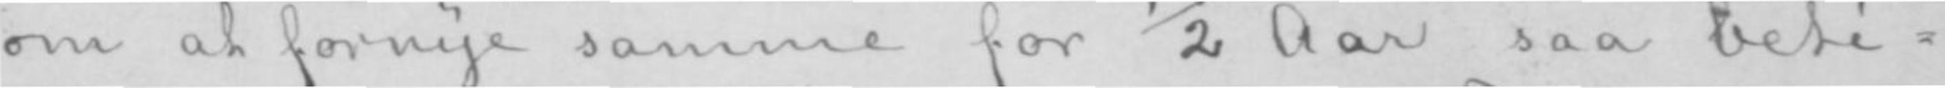om at fornye samme for 1/2 Aar saa beti-
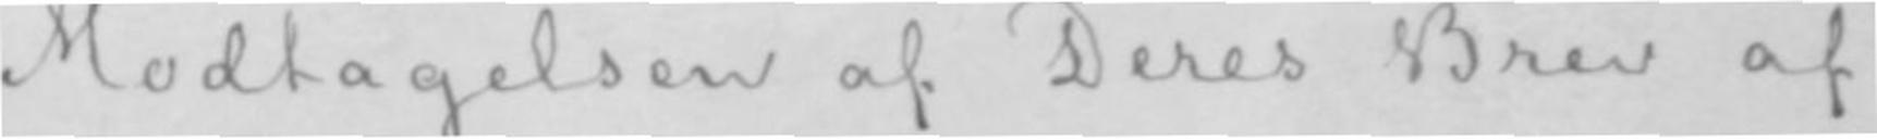Modtagelsen af Deres Brev af
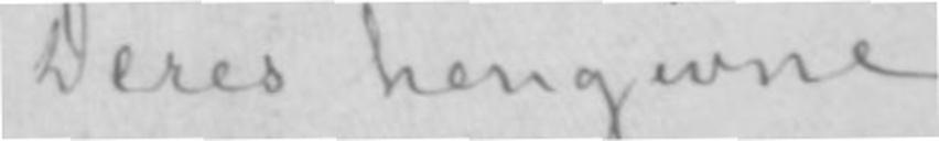Deres hengivne
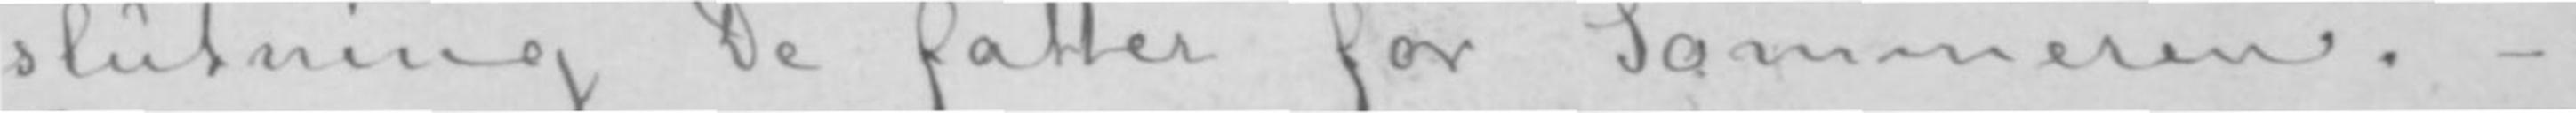slutning De fatter for Sommeren.-
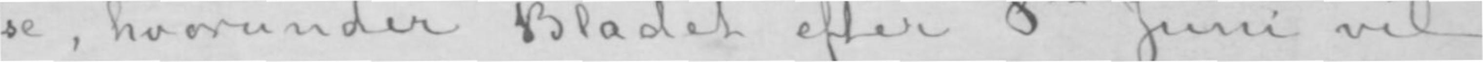se, hvorunder Bladet efter 8de Juni vil
